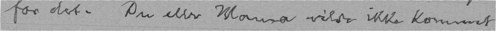for det. Du eller Mama vilde ikke kommet
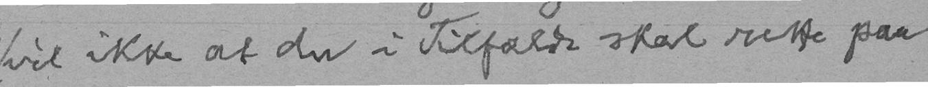vil ikke at du i Tilfælde skal rette paa
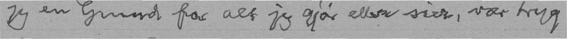jeg en Grund for alt jeg gjør eller sier, vær tryg
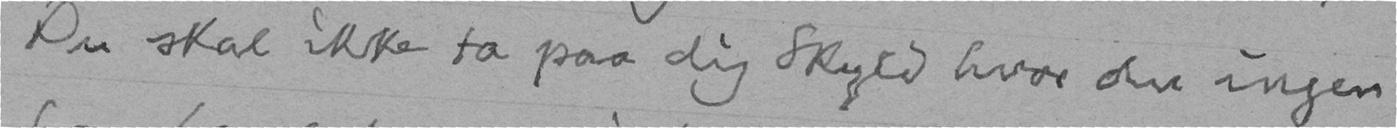Du skal ikke ta paa dig Skyld hvor du ingen
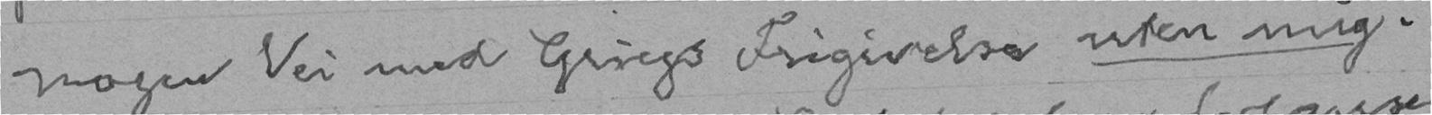nogen Vei med Griegs Frigivelse uten mig.
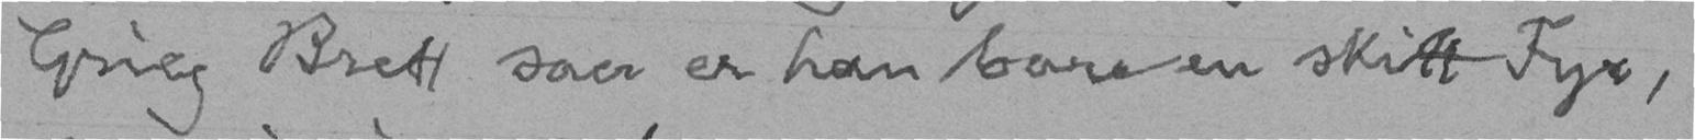Grieg Brett saa er han bare en skitt Fyr,
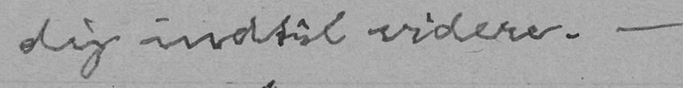dig indtil videre. -
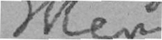Men
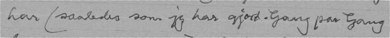har (saaledes som jeg har gjort. Gang paa Gang
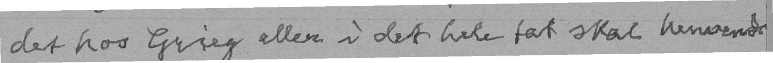det hos Grieg eller i det hele tat skal henvende
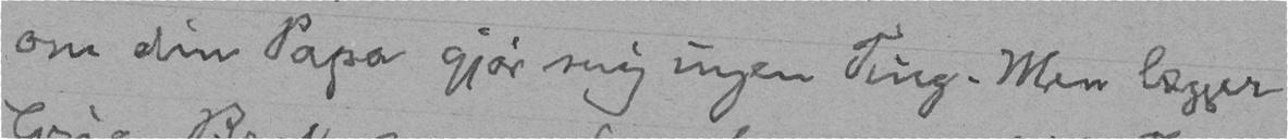om din Papa gjør mig ingen Ting. Men lægger
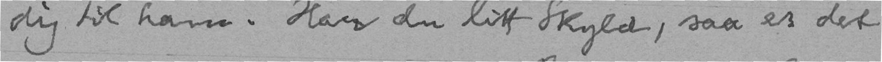dig til ham. Har du litt Skyld, saa er det
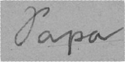Papa
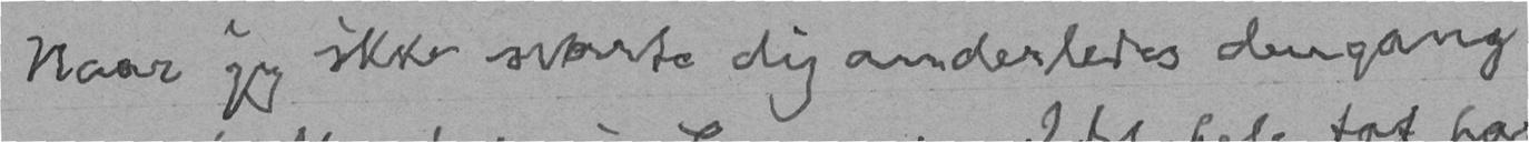Naar jeg ikke svarte dig anderledes dengang
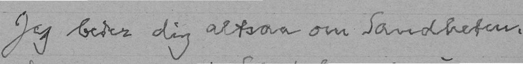Jeg beder dig altsaa om Sandheten.
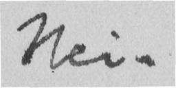Nei.
